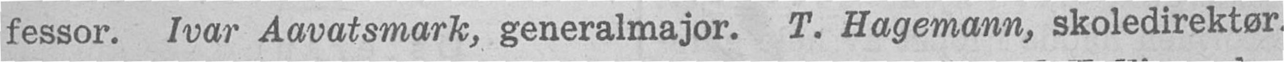fessor. Ivar Aavatsmark, generalmajor. T. Hagemann, skoledirektør.
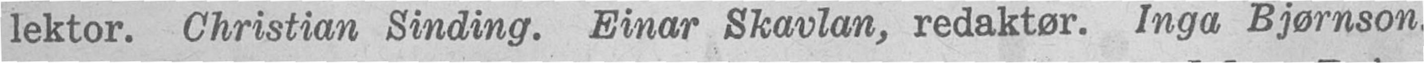lektor. Christian Sinding. Einar Skavlan, redaktør. Inga Bjørnson.
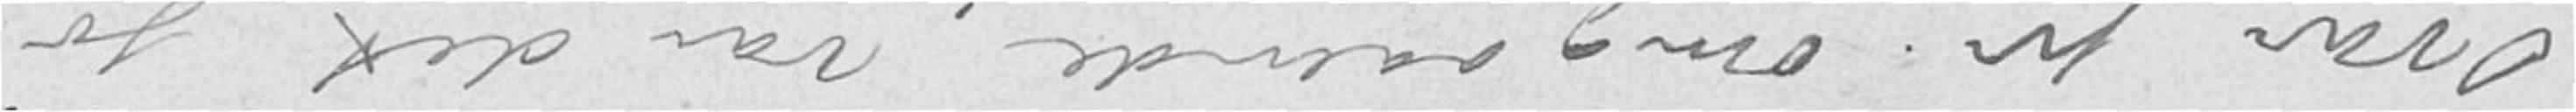svar pr. omgaaende, var det jo
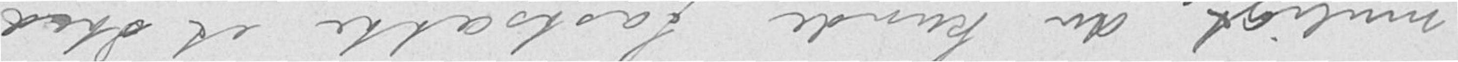muligt, du kunde fastsætte et sted
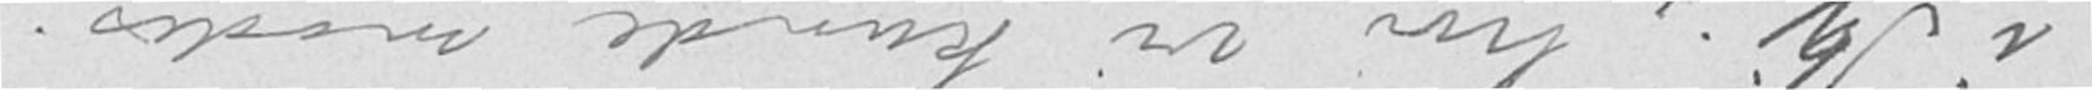i Kj., hvor vi kunde mødes.
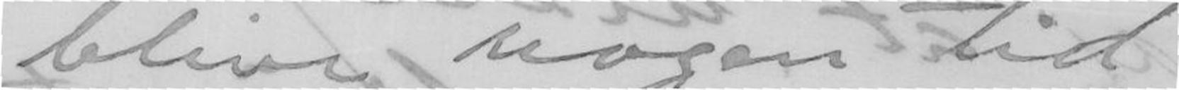blive nogen tid
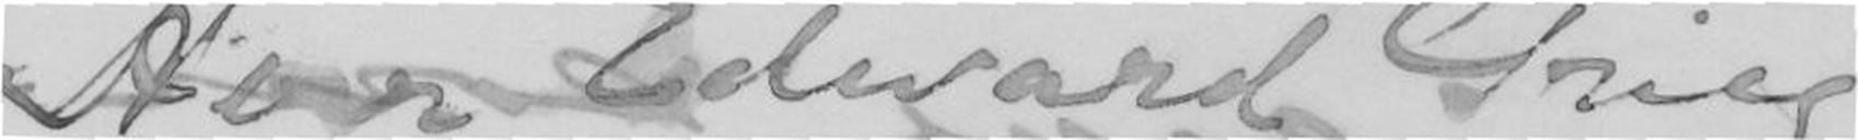Herr Edvard Grieg.
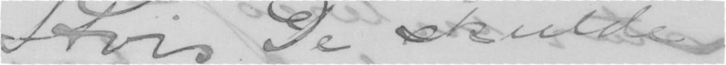Hvis De skulde
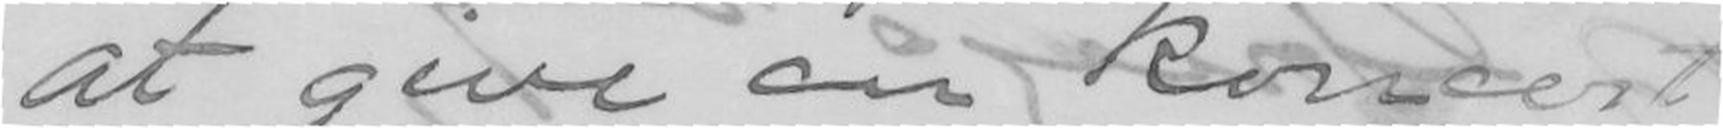at give en koncert
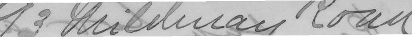93 Mildway Road
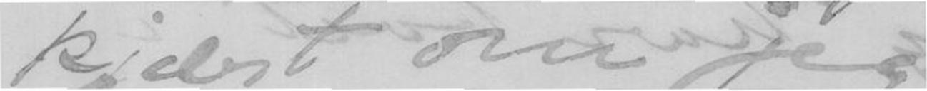kjært om jeg
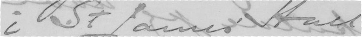i St James Hall.
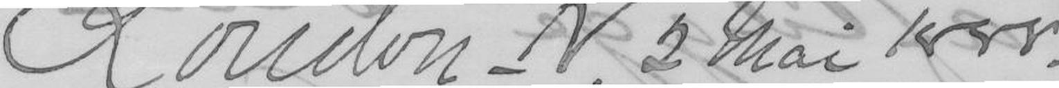London N. 2 Mai 1888.
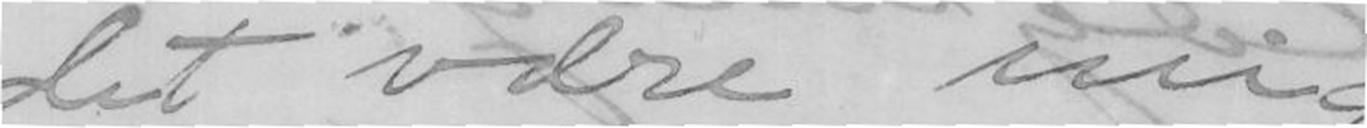det være mig
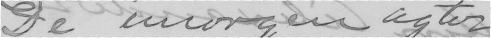De imorgen agter
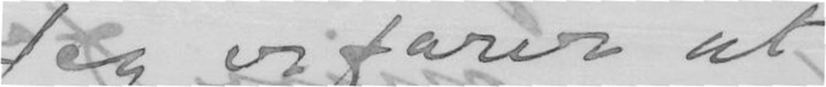Jeg erfarer at
